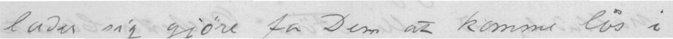lader sig gjøre for Dem at komme løs i
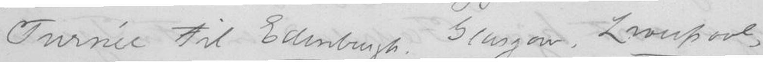Turnée til Edenburgh Glasgow, Liverpool,
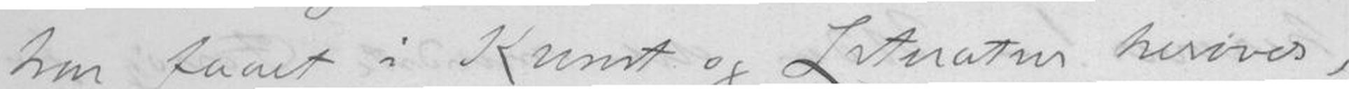har faaet i Kunst og Literatur herover,
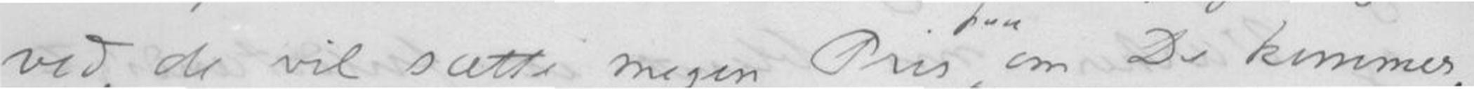ved, de vil sætte megen Pris paa om De kommer,
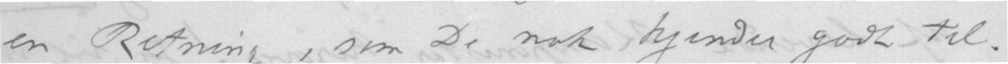en Retning, som De nok kjender godt til.
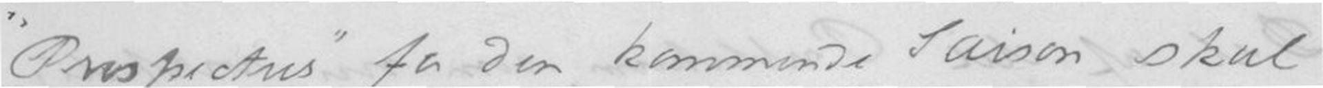"Prospectus" for den kommende Saison skal
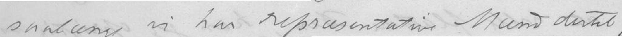saalænge vi har repræsentative Mand dertil,
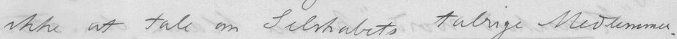ikke at tale om Selskabets talrige Medlemmer.
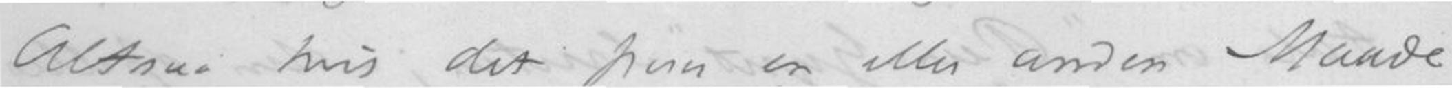Altsaa hvis det paa en eller anden Maade
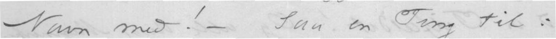Navn med! - Saa en Ting til:
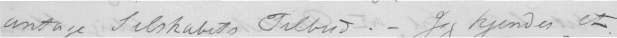antage Selskabets Tilbud. - Jeg kjender et
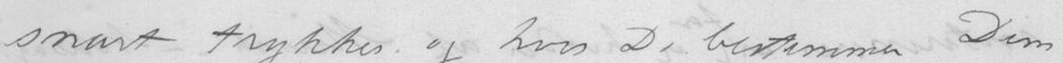snart trykkes, og hvis De bestemmer Dem
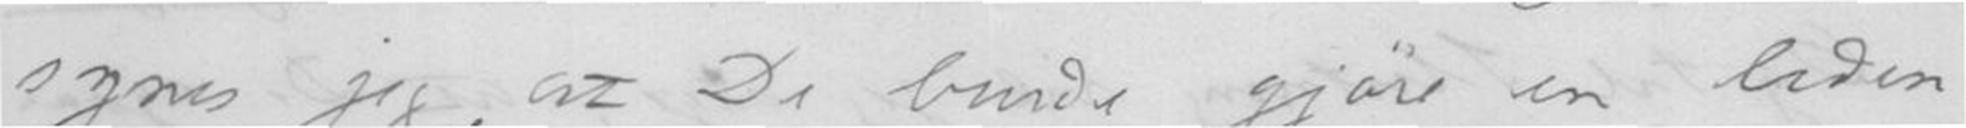synes jeg, at De burde gjøre en liden
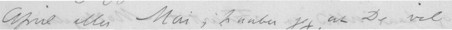April eller Mai, haaber jeg, at De vil
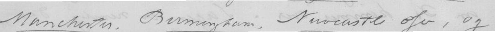Manchester, Birmingham, Newcastle osv, og
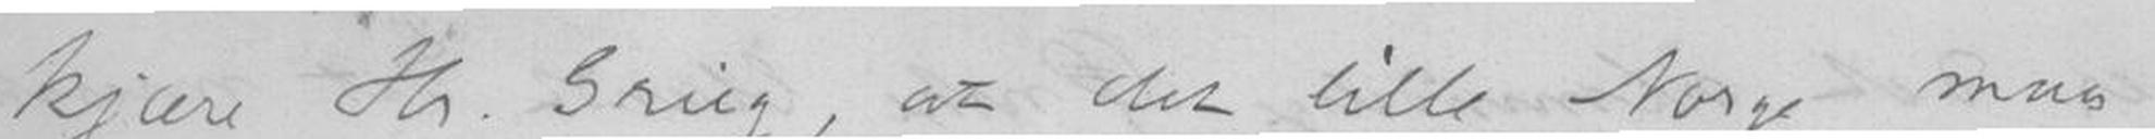kjære Hr. Grieg, at det lille Norge maa
